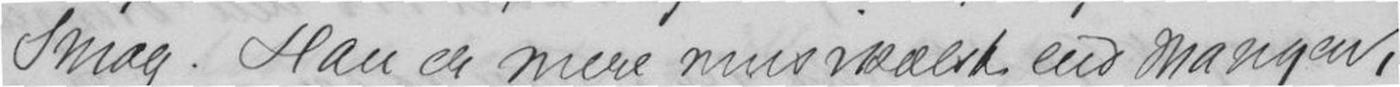Smag. Han er mere musikalsk end mangen,
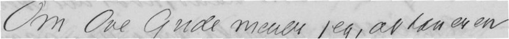Om Ove Gude mener jeg, at han er en
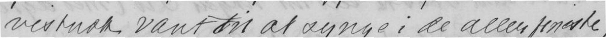vistnok vant til at synge i de aller fineste,
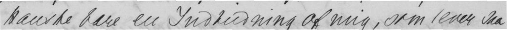kanske bare en Indbudning af mig, som lever saa
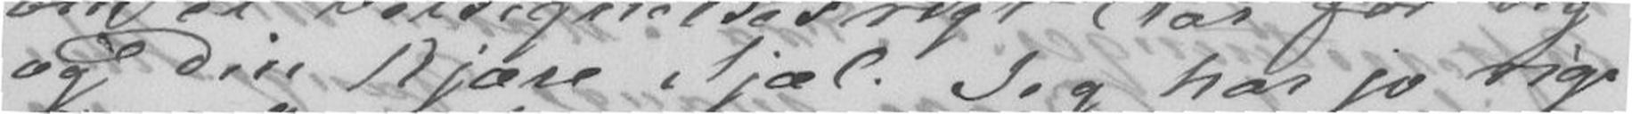og Din kjære Sjæl. Jeg har jo rig-
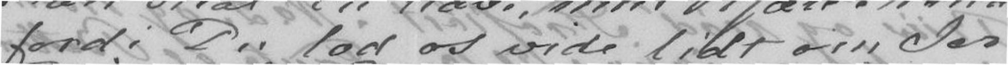fordi Du lod os vide lidt om Jer
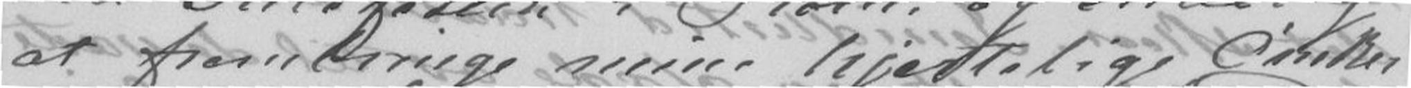at frembringe mine hjertelige Ønsker
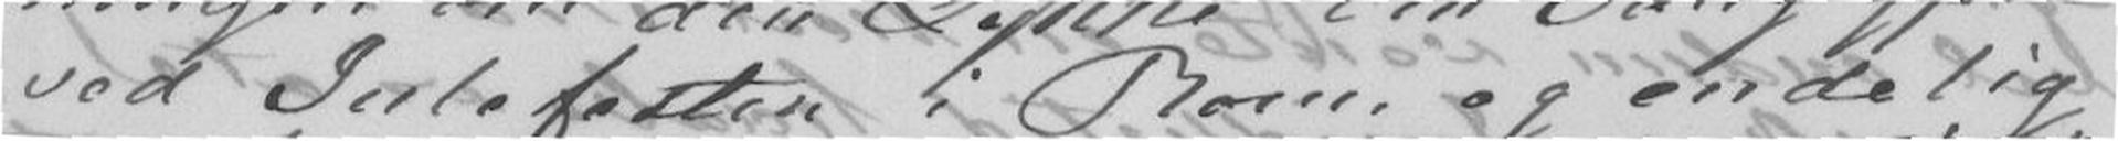ved Julefesten i Rom og endelig
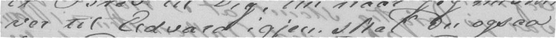ver til Edvard igjen skal Du ogsaa
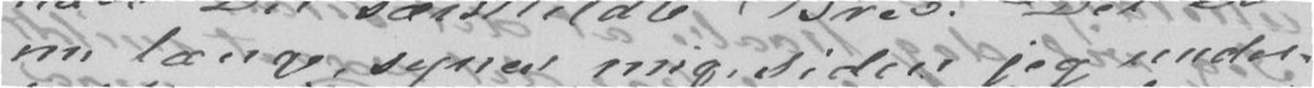nu længe, synes mig, siden jeg under-
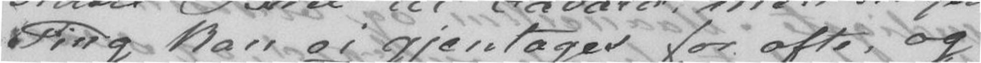Ting kan ei gjentages for ofte, og
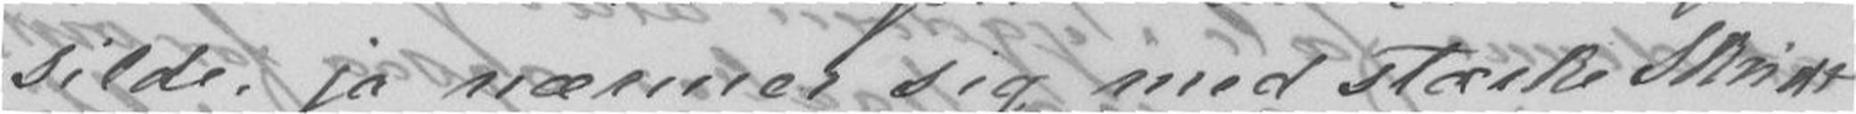silde, jo nærmere sig med stærke Skridt
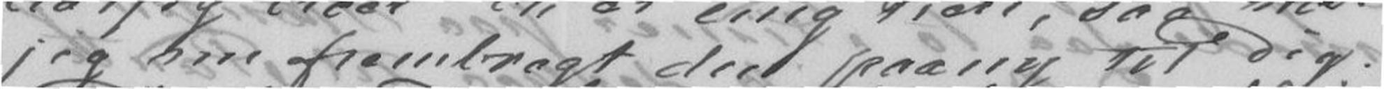jeg nu frembragt den paany til Dig.
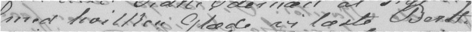med hvilken Glæde vi læste Beret-
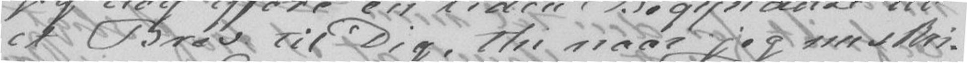et Brev til Dig, thi naar jeg nu skri-
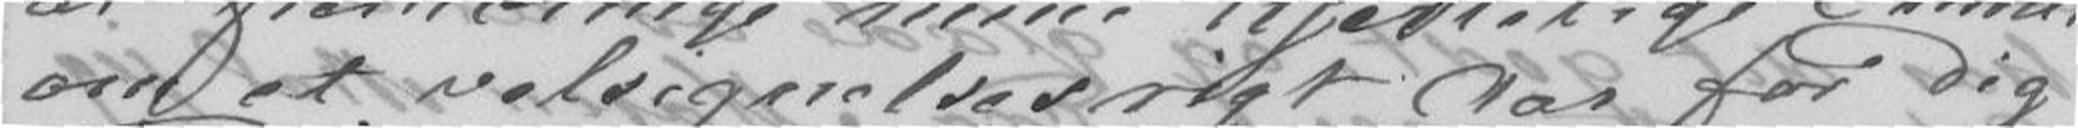om et velsignelsesrigt Aar for Dig
